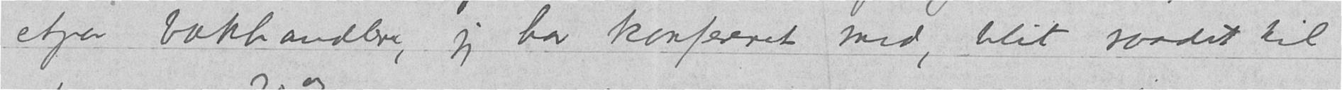etpar bokhandlere, jeg har konfereret med, blit raadet til
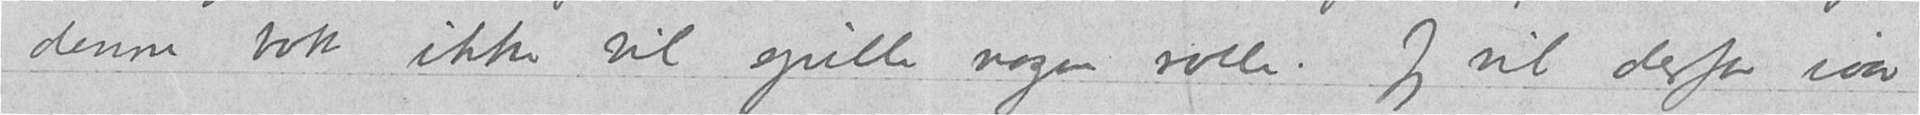denne bok ikke vil spille nogen rolle. Jeg vil derfor iaar
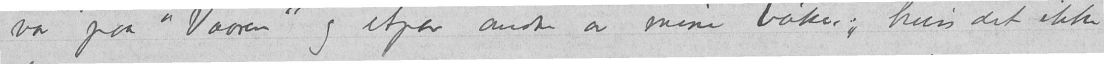var paa "Vaaren" og etpar andre av mine bøker; hvis det ikke
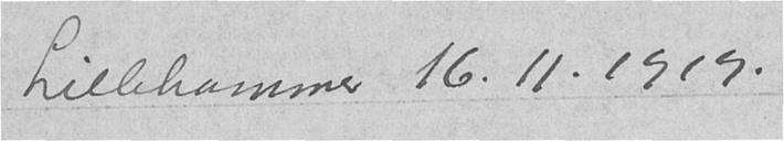Lillehammer 16.11.1919.
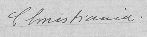Christiania.
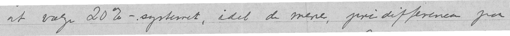at vælge 20%-systemet, idet de mener, prisdifferencen paa
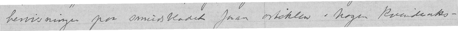henvisninger paa smudsbladet foran artiklen i "Nogen Kvindesaks-
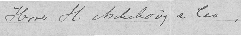Herrer H. Aschehoug & Co,
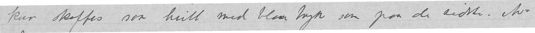kan skaffes saa hvitt med blaa tryk som paa de sidste. Av
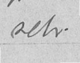selv.
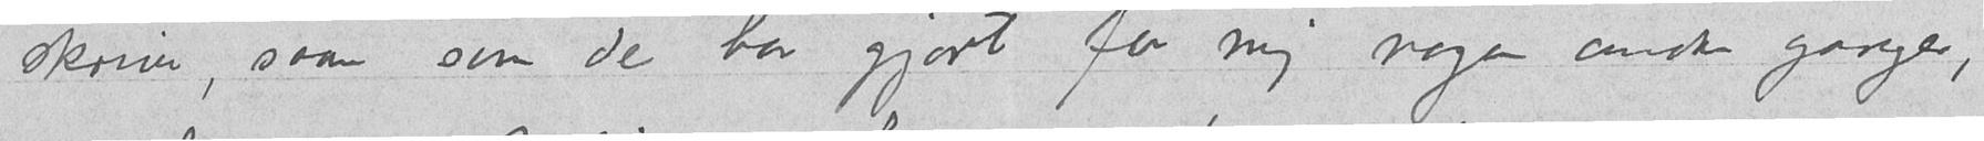skrive, saan som de har gjort for mig nogen andre ganger,
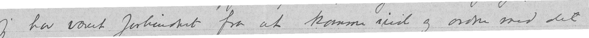jeg har været forhindret fra at komme ind og ordne med det
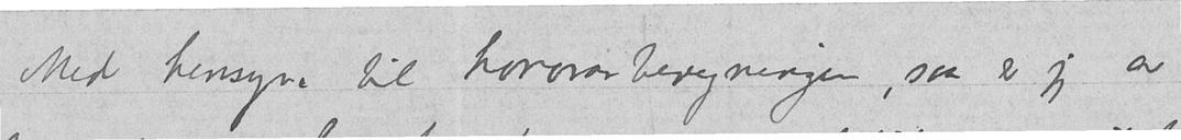Med hensyn til honorarberegningen, saa er jeg av
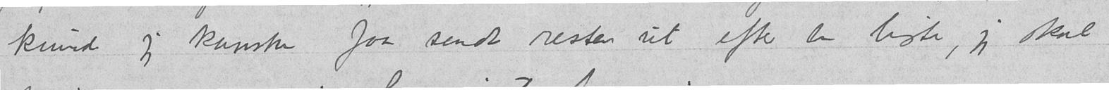kunde jeg kanske faa sendt resten ut, efter en liste, jeg skal
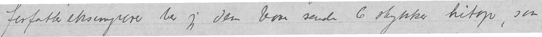forfattereksemplarer ber jeg dem bare sende 6 stykker hitop, saa
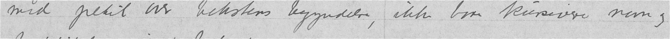med petit over tekstens begyndelse, ikke bare kursivere navn og
